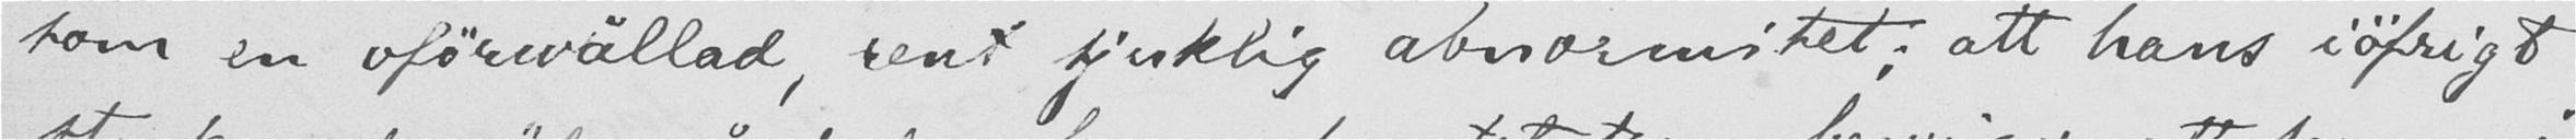som en oførwällad, rent sjuklig abnormitet; att hans iøfrigt
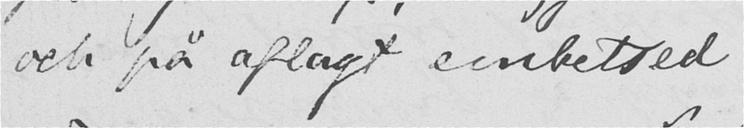och på aflagt embetsed
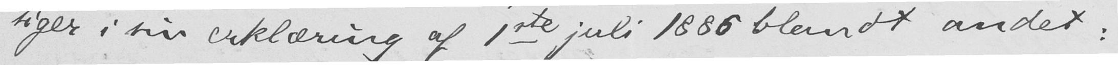siger i sin erklæring af 1ste juli 1886 blandt andet:
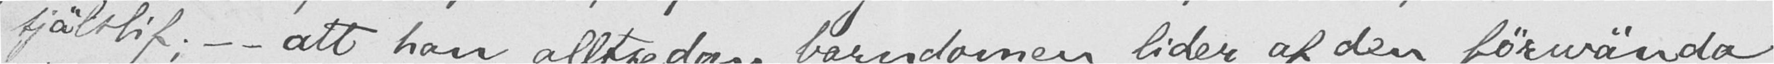själslif; - - att han alltsedan barndomen lider af den førwända
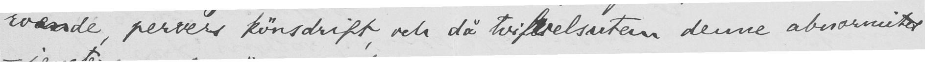roende, pervers kønsdrift, och då tvifrelsutem denne abnormitet
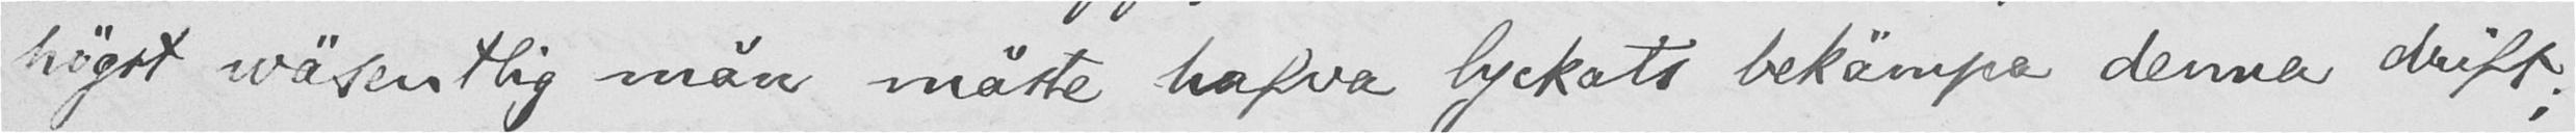høgst wäsentlig mån måste hafva lyckats bekämpa denna drift;
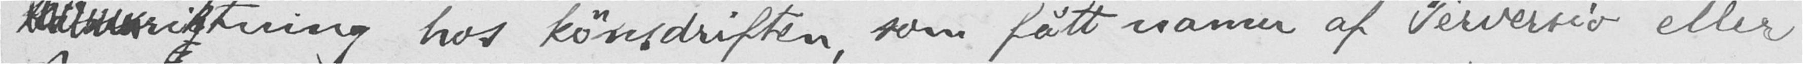kauriftning hos kønsdriften, som fått namn af Perversio eller
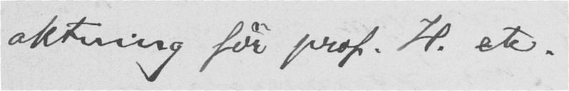aktning før prof. H. etc. -
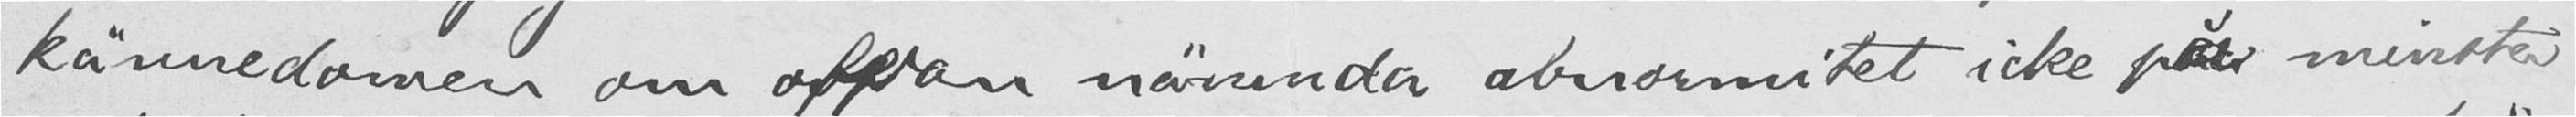kännedomen om offran nämda abnormitet icke på minste
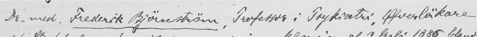Dr. med. Frederik Bjørnstrøm, Professor i Psykiatri, Ofverläkare
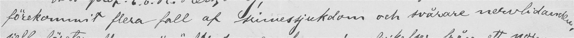førekommit flera fall af sinnessjukdom och svårare nervlidander,
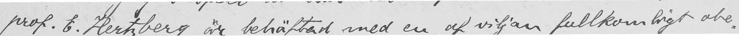prof. E. Hertzberg är behäftad med en af viljan fullkomligt obe-
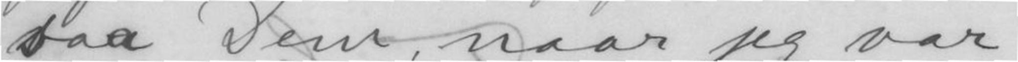saa Dem, naar jeg var
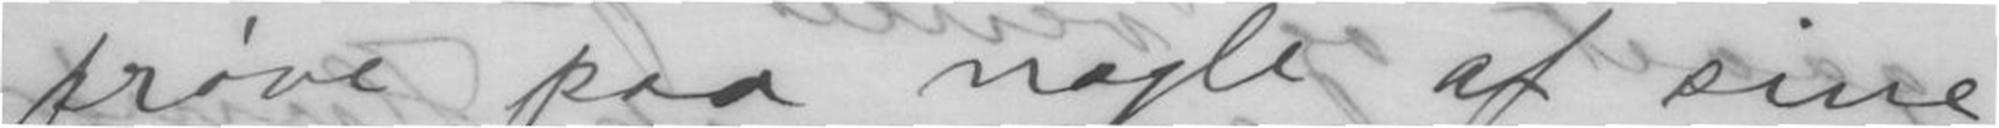prøve paa nogle af sine
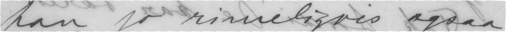han jo rimeligvis ogsaa
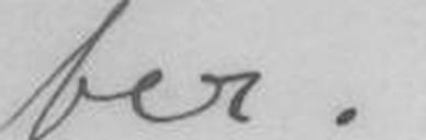ber.
